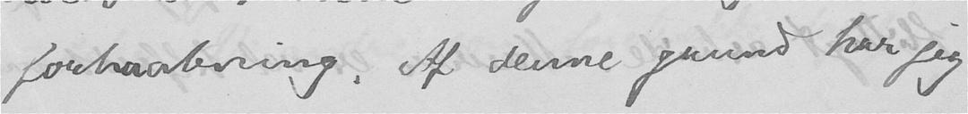forhaabning. Af denne grund har jeg
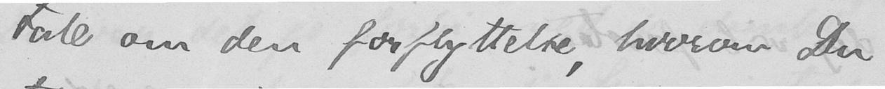tale om den forflyttelse, hvorom Du
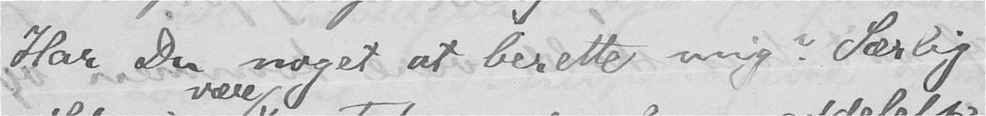Har Du noget at berette mig? Særlig
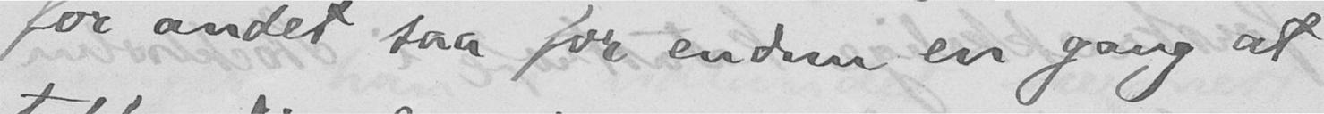for andet saa for endnu en gang at
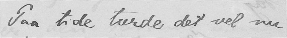Paa tide turde det vel nu
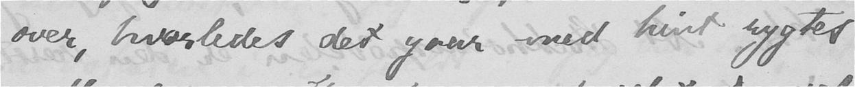over, hvorledes det gaar med hint rygtes
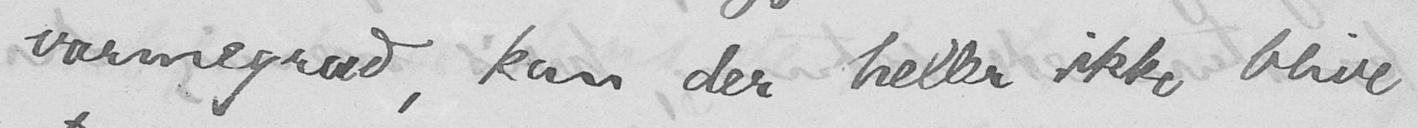varmegrad, kan der heller ikke blive
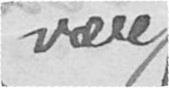være
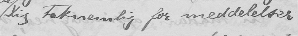Dig taknemlig for meddelelser
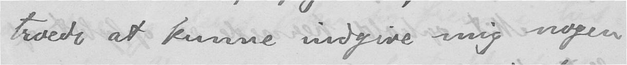troede at kunne indgive mig nogen
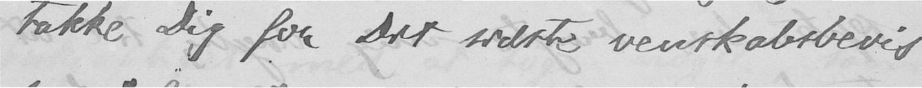takke Dig for Dit sidste venskabsbevis
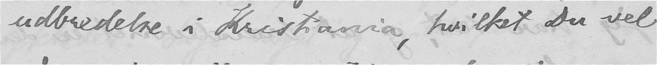udbredelse i Kristiania, hvilket Du vel
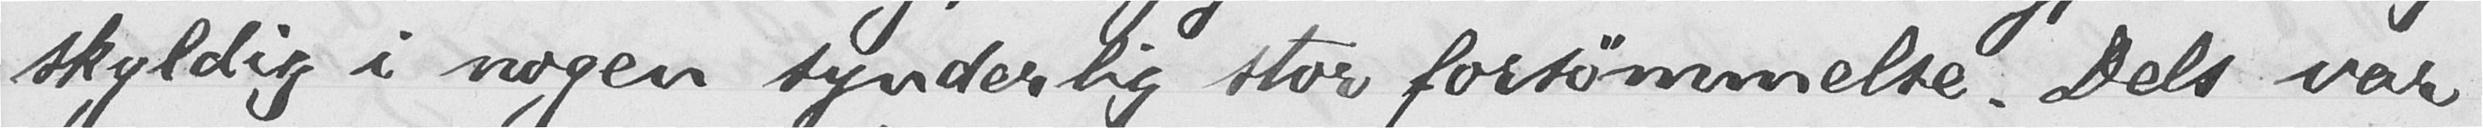skyldig i nogen synderlig stor forsømmelse. Dels var
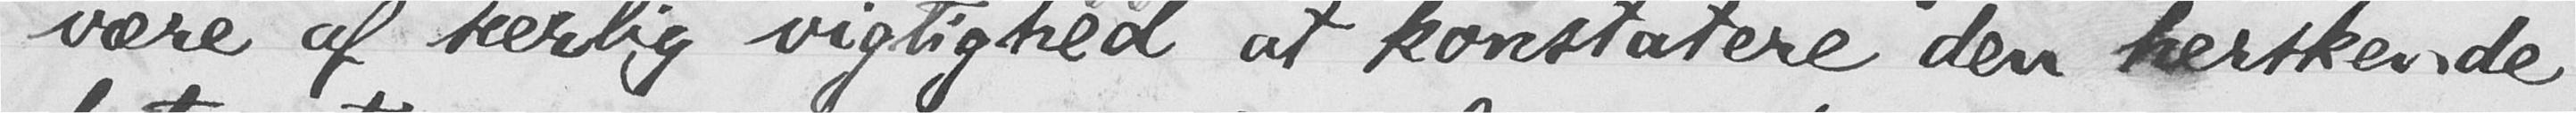være af særlig vigtighed at konstatere den herskende
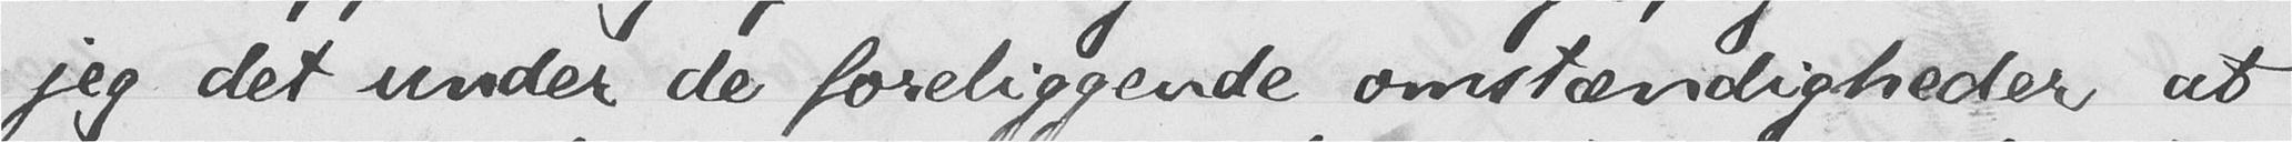jeg det under de foreliggende omstændigheder at
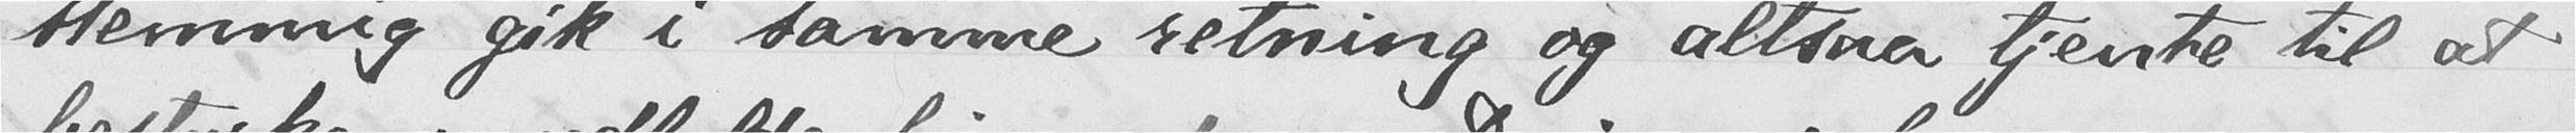stemmig gik i samme retning og altsaa tjente til at
